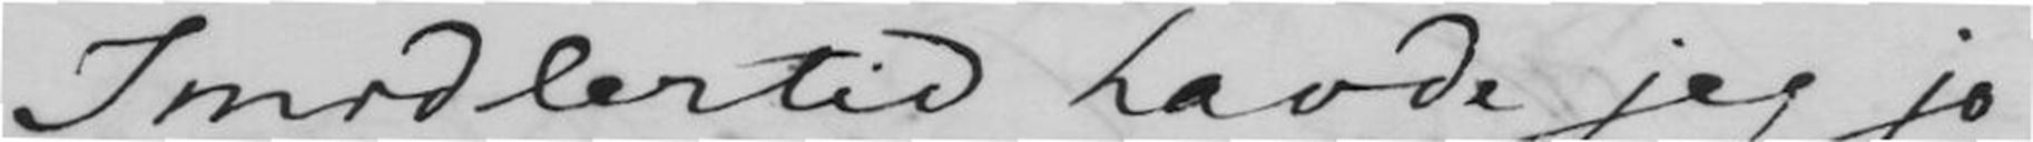Imidlertid havde jeg jo
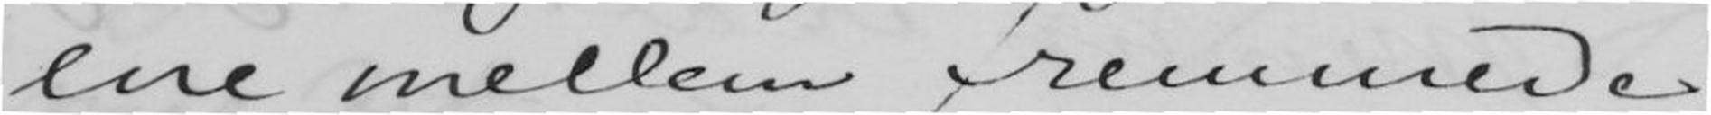ene mellem fremmede
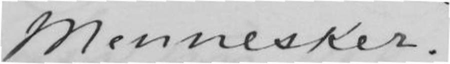Mennesker.
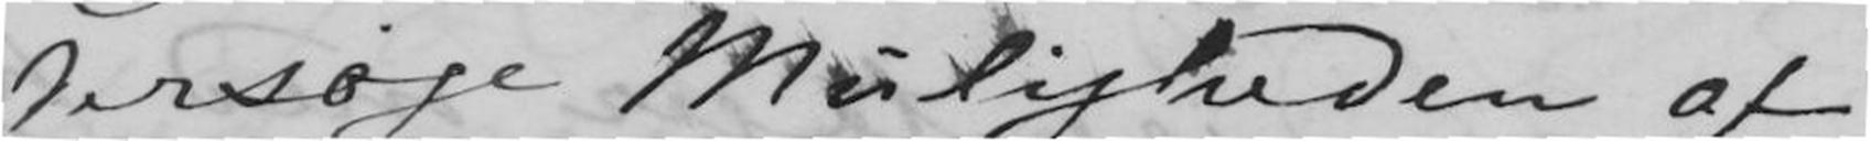dersøge Muligheden af
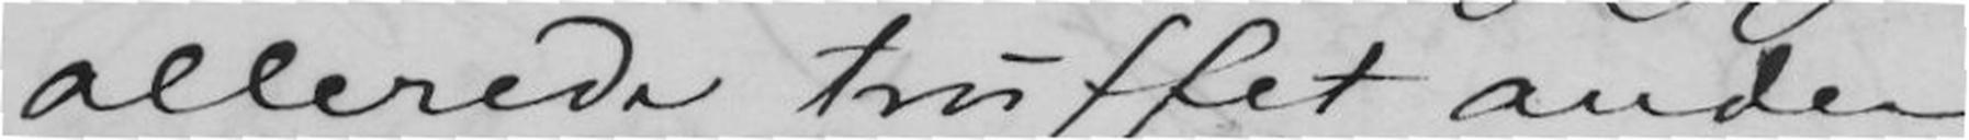allerede truffet anden
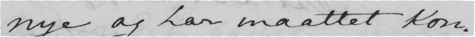nye og har maattet Kon-
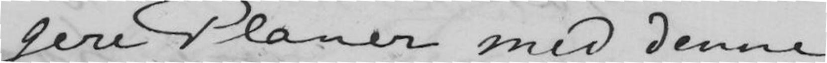gere Planer med denne
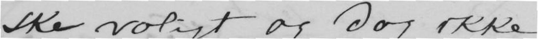ske roligt og Dog ikke
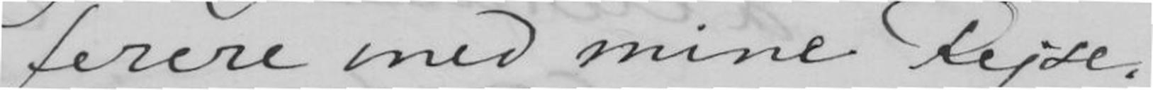ferere med mine Rejse-
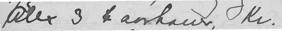Alex 3 aarhaner, Kr.
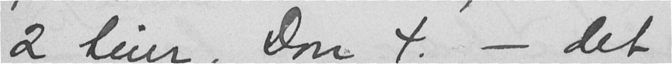2 tiur, Don 4. - det
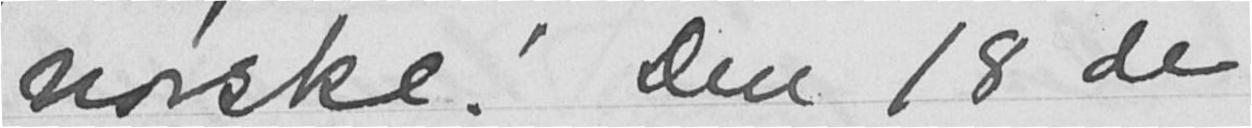norske! Den 18 de
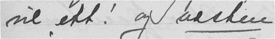vil ett! og næsten
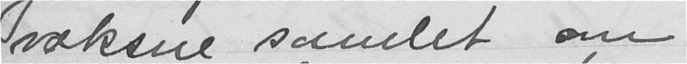voksne samlet om
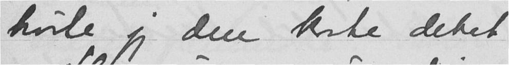hørte jeg den korte debat
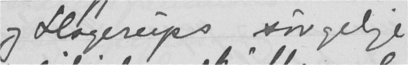og Hagerups sørgelige
